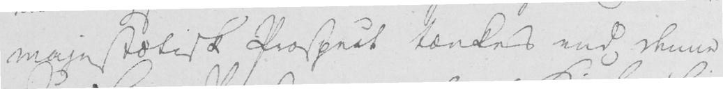majestætisk Prospect tænkes end denne
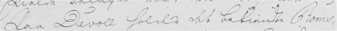Paa Devoll holdes det bekiendte Roms-
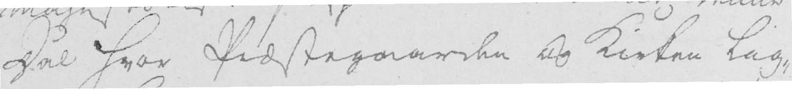Dal hvor Præstegaarden og Kirken lig-
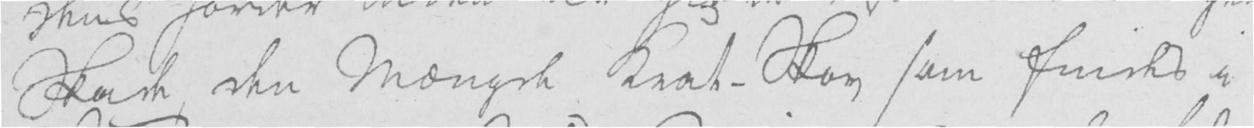Skade, den Mængde Krat-Skov som findes i
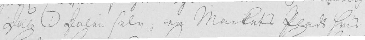Dal i Dalen selv; en Markets Plads hvis
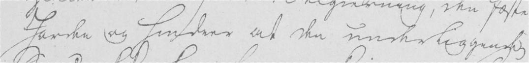Jorden og hindrer at den underliggende
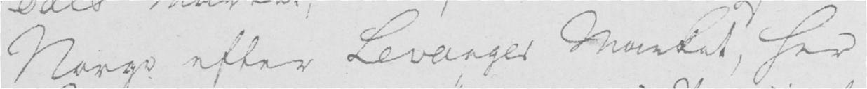Norge efter Levanger Market, her
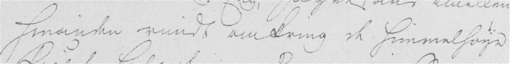hinanden rundt omkring de himmelhøye
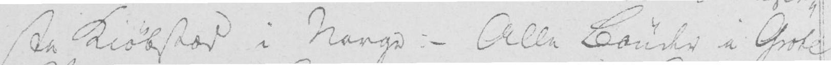ste Kiøbstad i Norge. - Alle Bønder i Grote
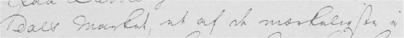dals Market, et af de mærkeligste i
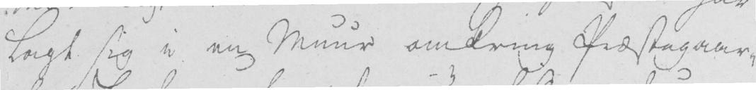lagt sig i en Muur omkring Præstegaar-
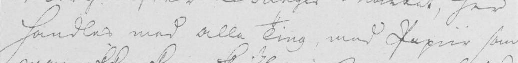handles med alle Ting, med Papir som
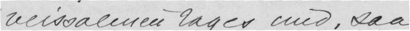veissalmen tages med, saa
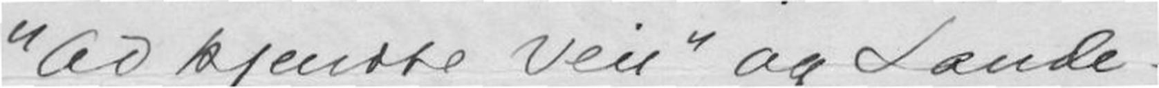"Av kjendte Veil" og Lande-
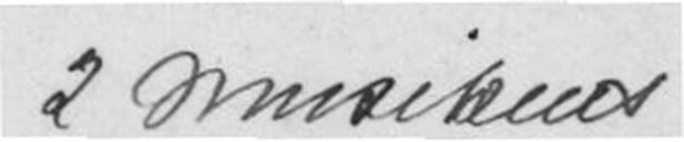2 musikens
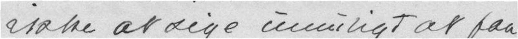ikke at sige umuligt at faa
